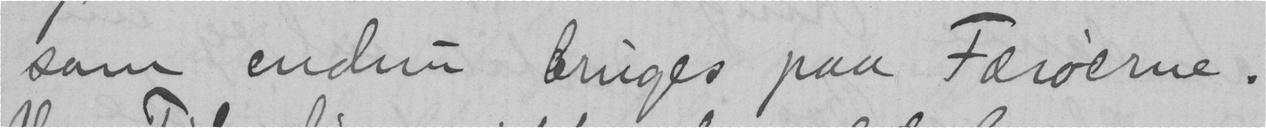som endnu bruges paa Færøerne.
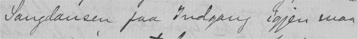Sangdansen faa Indgang igjen maa
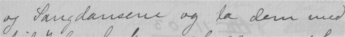og Sangdansene og ta dem med
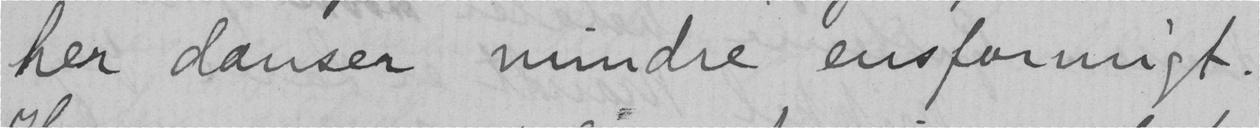her danser mindre ensformigt.
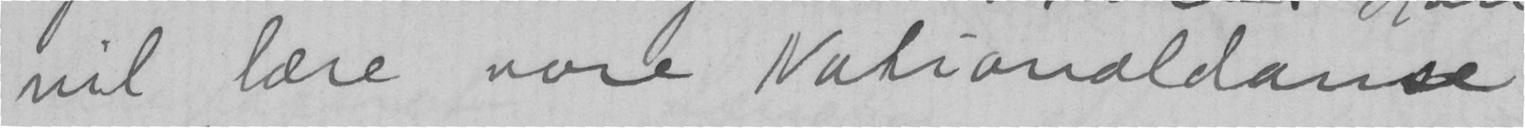vil lære vore Nationaldanse
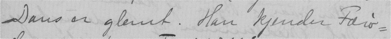Dans er glemt. Han kjender Færø-
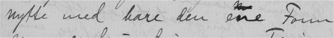nytte med bare den ene Form
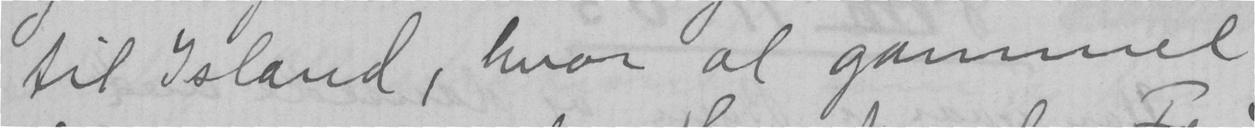til Island, hvor al gammel
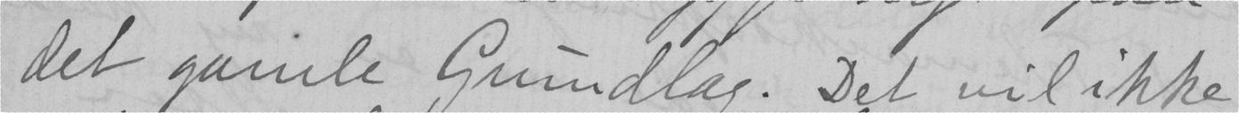det gamle Grundlag. Det vil ikke
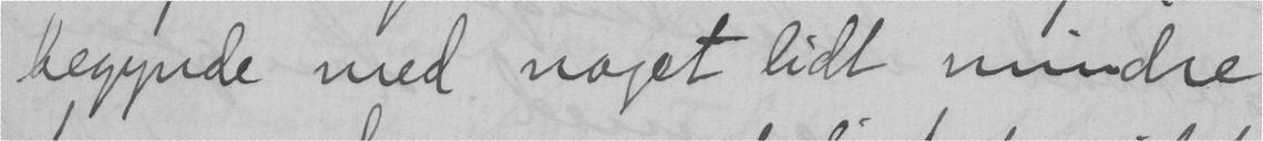begynde med noget lidt mindre
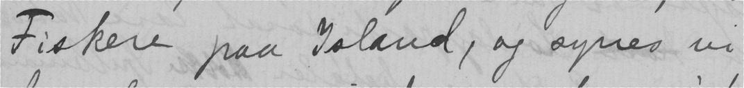Fiskere paa Island, og synes vi
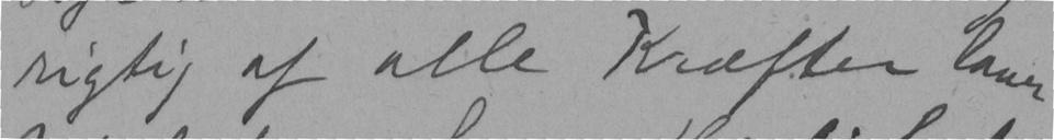rigtig af alle Kræfter laver
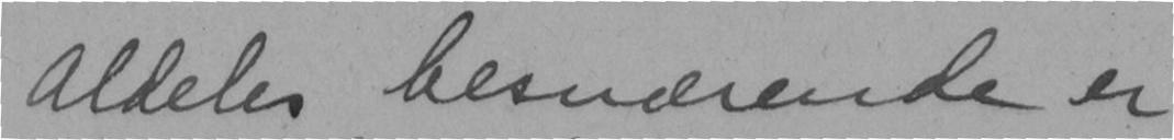Aldeles besnærende er
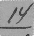14
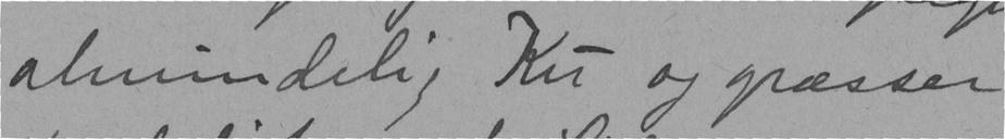almindelig Ku og græsser
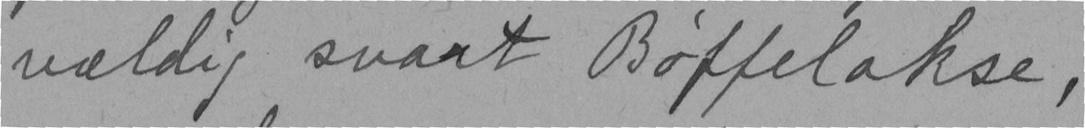vældig svart Bøffelokse,
